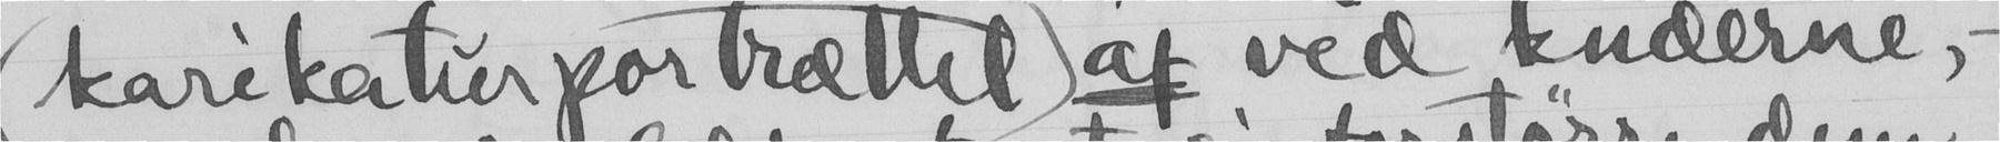(karikaturportrættet) af ved knæerne, -
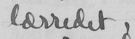lærredet,
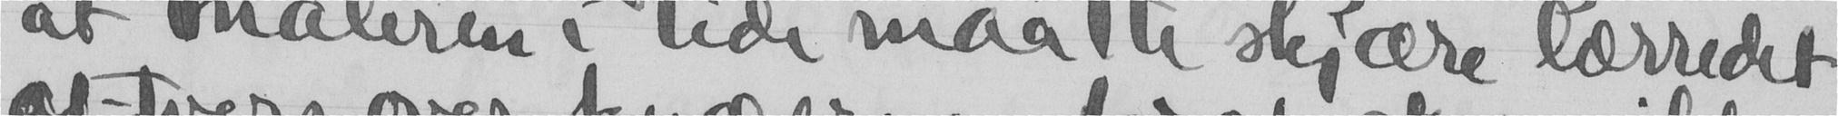at maleren i tide maatte skjære lærredet
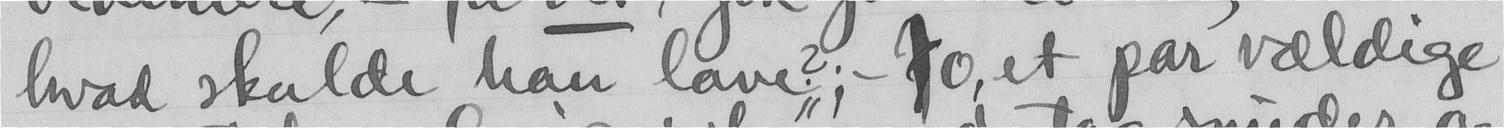hvad skulde han lave?; - Jo, et par vældige
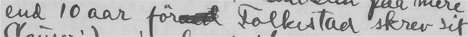end 10 aar før Folkestad skrev sit
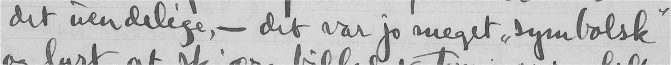det uendelige, - det var jo meget "symbolsk"
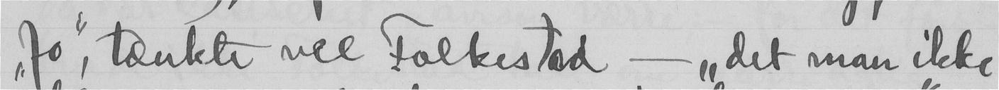"Jo," tænkte vel Folkestad - "det man ikke
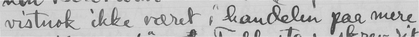vistnok ikke været i handelen paa mere
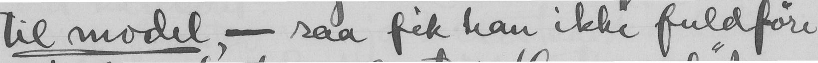til model - saa fik han ikke fuldføre
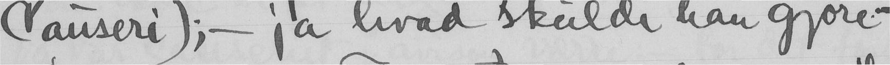Causeri); - ja hvad skulde han gjøre? -
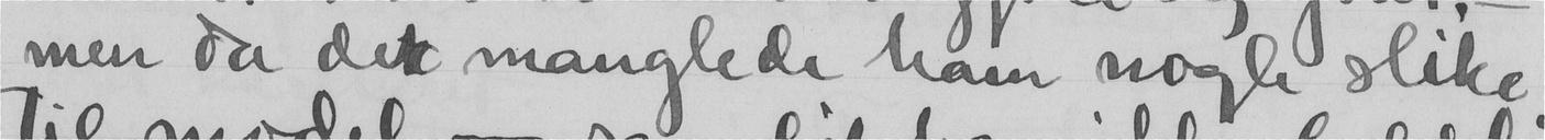men da det manglede ham nogle slike
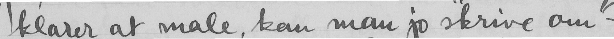klarer at male, kan man jo skrive om"-
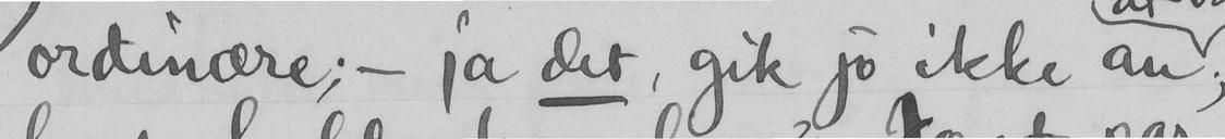ordinære; - ja det, gik jo ikke an
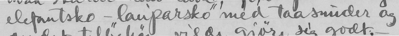elefatsko - "lauparsko" med taasnuder og
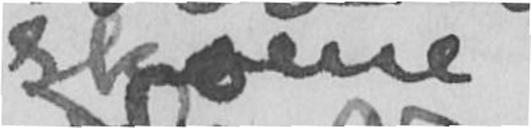skoene
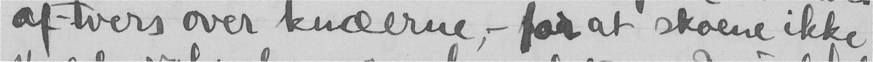af tvers over knæerne, - far at skoene ikke
